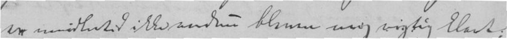er imidlertid ikke endnu bleven mig rigtig klart;
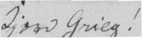Kjære Grieg!
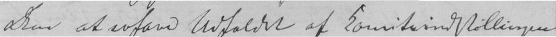Dem at erfare Udfaldet af Komiteindstillingen
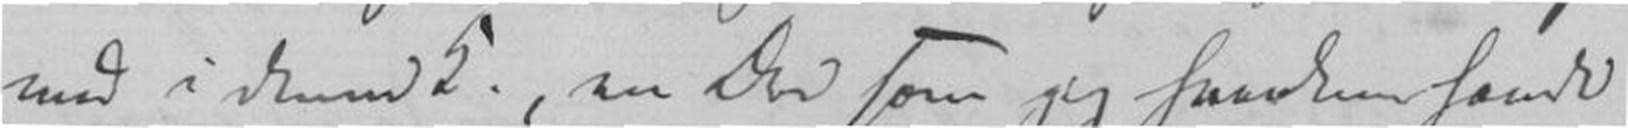med i denne K., en Der som jeg saadan havde
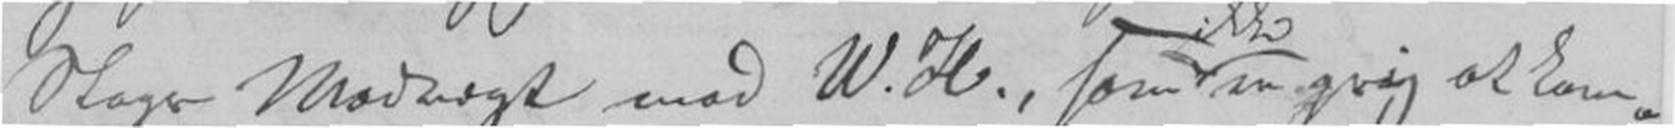Slags Modvægt mod W.H., som er grej at kom-
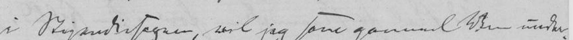i Stipendiesagen, vil jeg som gammel Ven under-
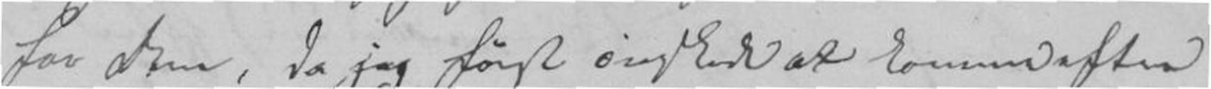for Den, da jeg først ønskede at komme efter
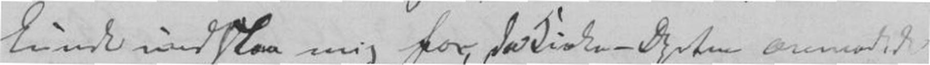kunde indstaa mig for, da Kirken-Dysten anmodede
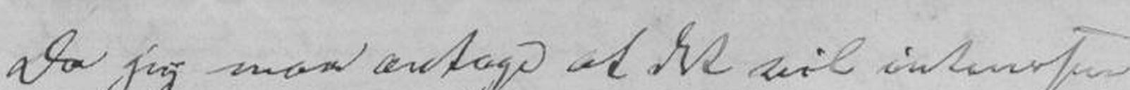Da jeg maa antage at det vil interessere
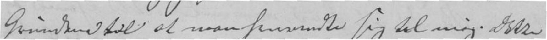Grunden til at man henvendte sig til mig. Dette
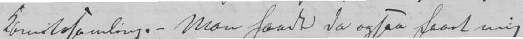Komitesamling. - Man havde da ogsaa faaet mig
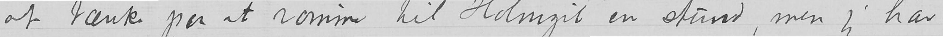at tænke paa at rømme til Holmgil en stund, men jeg har
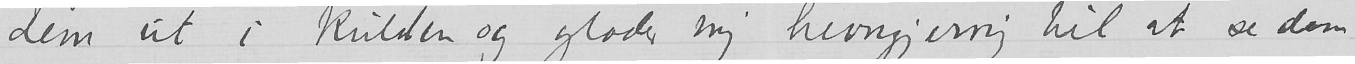dem ut i kulden og glæder mig hevngjerrig til at se dem
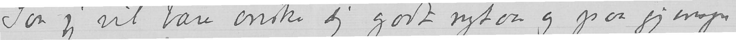Saa jeg vil bare ønske dig godt nytaar og paa gjensyn
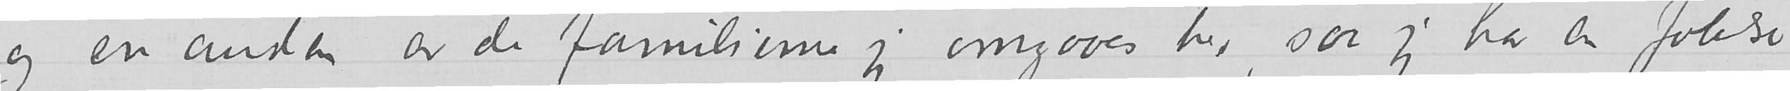og en anden av de familierne jeg omgaaes her, saa jeg har en følelse
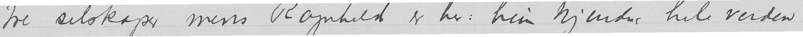tre selskaper mens Ragnhild er her: hun kjender hele verden
